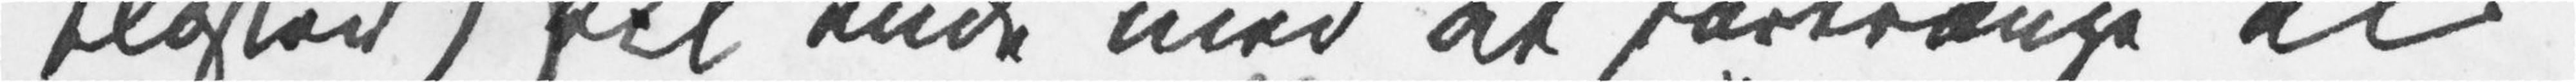Klasser) vil ende med at fortrænge al
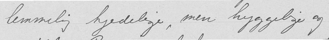temmelig kjedelige, men hyggelige og
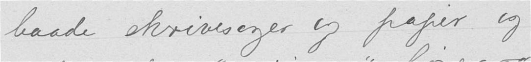baade skrivesager og papir og
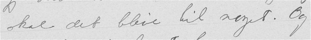skal det blive til noget. Og
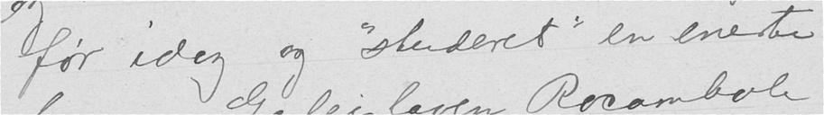før idag og "studeret" en eneste
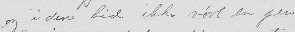og i den tid ikke rørt en pen
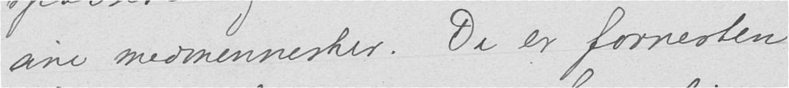sine medmennesker. De er forresten
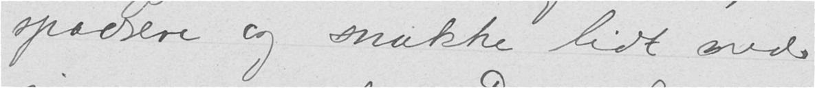spadsere og snakke lidt med
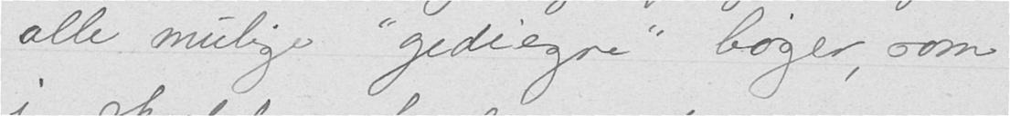alle mulige "gediegne" bøger, som
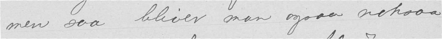men saa bliver man ogaaa noksaa
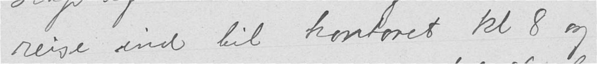reise ind til kontoret kl 8 og
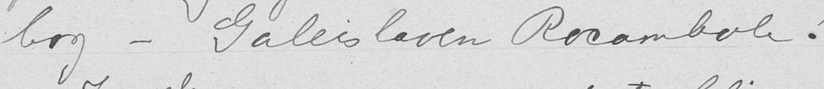bog - Galeislaven Rocambole!
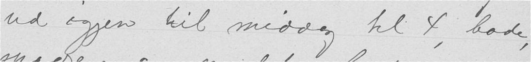ud igjen til middag kl 4, bade,
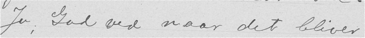Ja, Gud ved naar det bliver
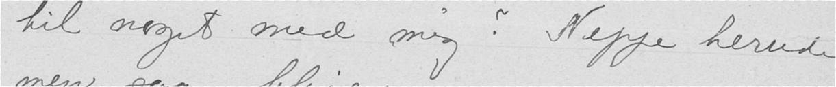til noget med mig? Neppe herude
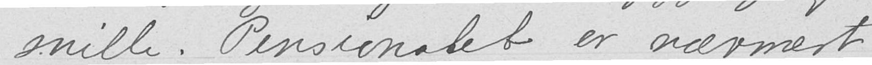snille. Pensionatet er nærmest
